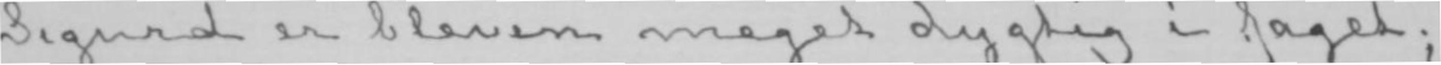Sigurd er bleven meget dygtig i faget;
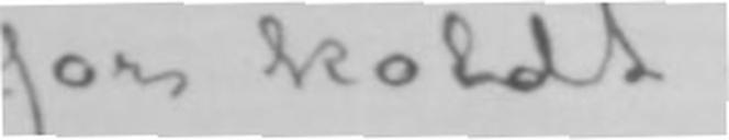for koldt.
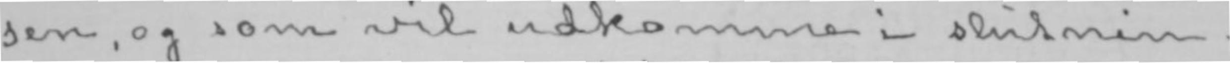sen, og som vil udkomme i slutnin-
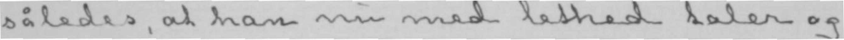således, at han nu med lethed taler og
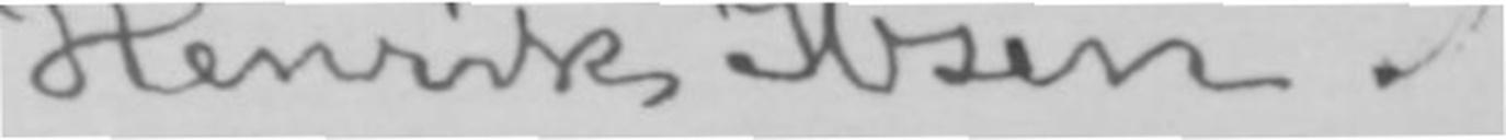Henrik Ibsen.
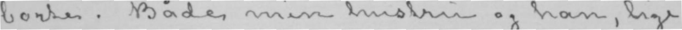borte. Både min hustru og han, lige-
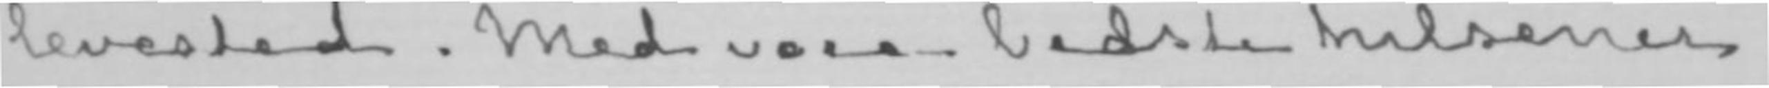levested. Med vore bedste hilsener
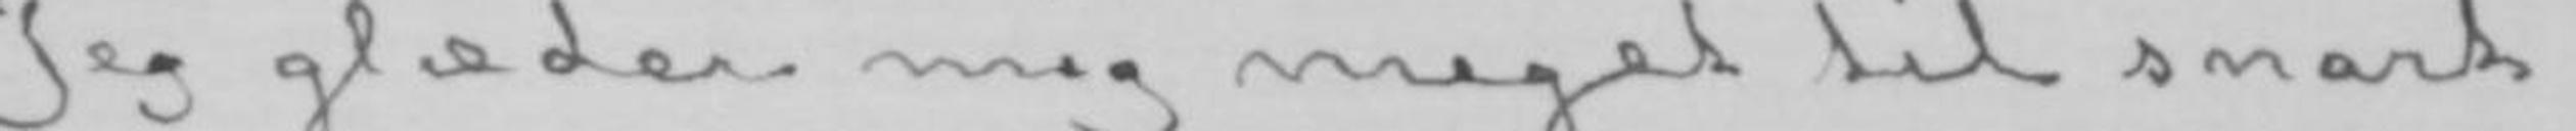Jeg glæder mig meget til snart
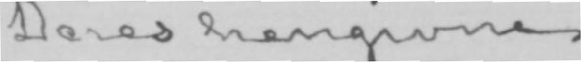Deres hengivne
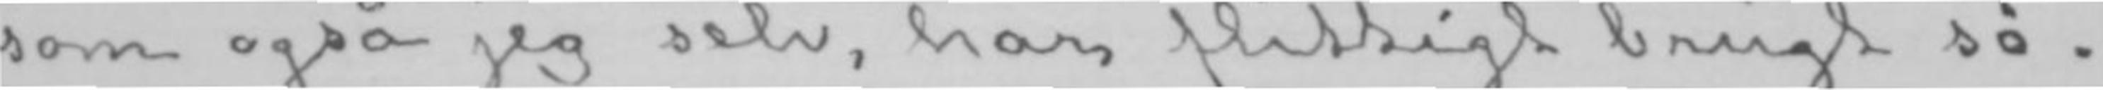som også jeg selv, har flittigt brugt sø-
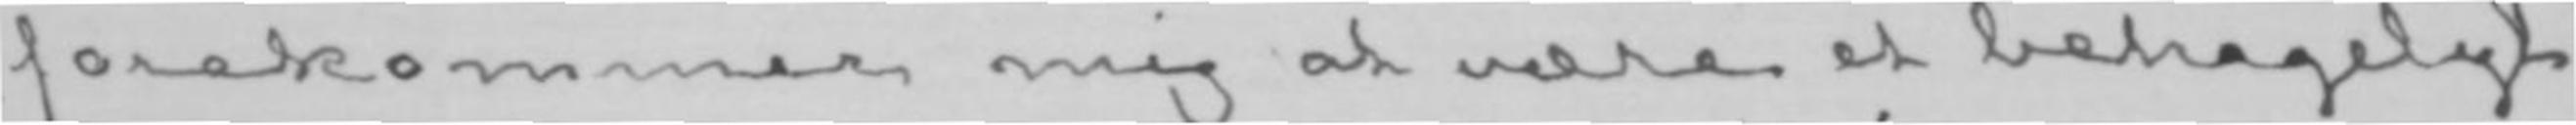forekommer mig at være et behageligt
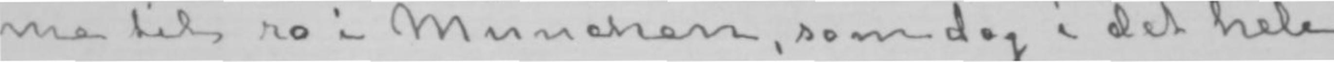me til ro i München, som dog i det hele
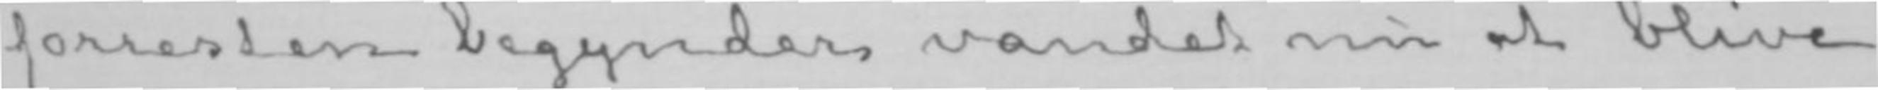forresten begynder vandet nu at blive
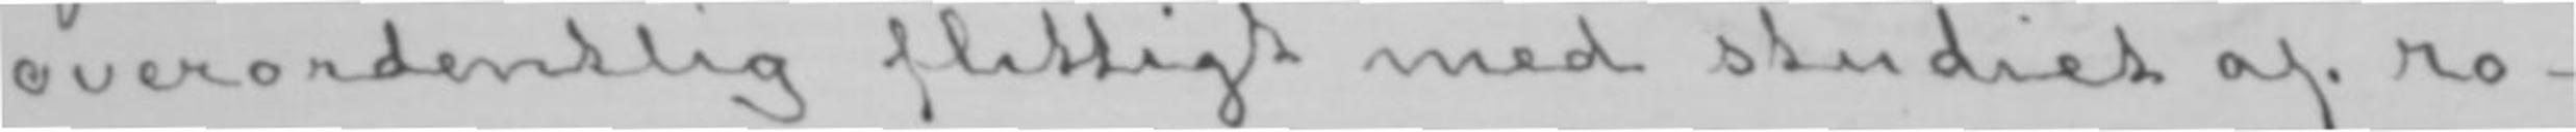overordentlig flittigt med studiet af ro-
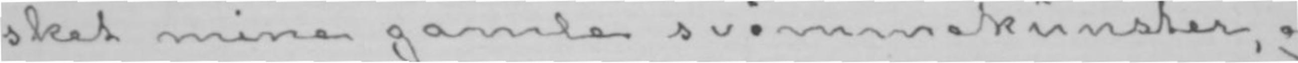sket mine gamle svømmekunster, og
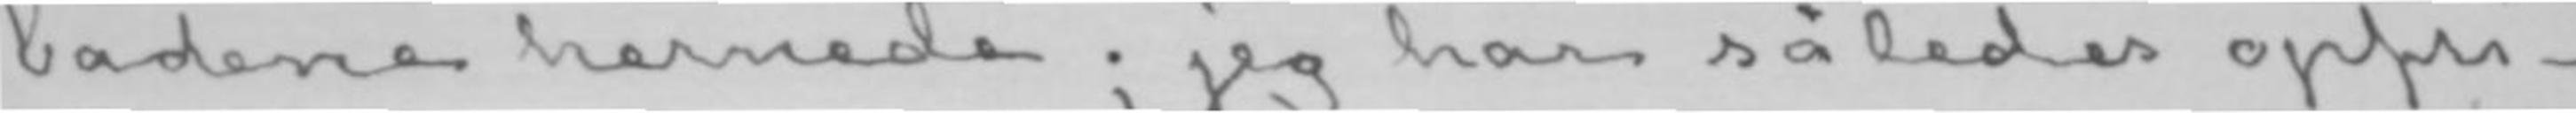badene hernede; jeg har således opfri-
